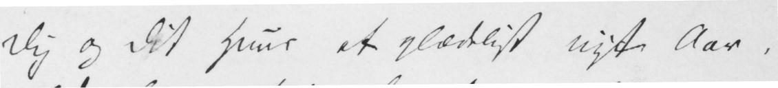Dig og Dit Huus et glædeligt nyt Aar,
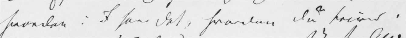hvordan: I have det, hvordan Du trives i
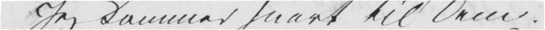Jeg kommer snart til Dem.
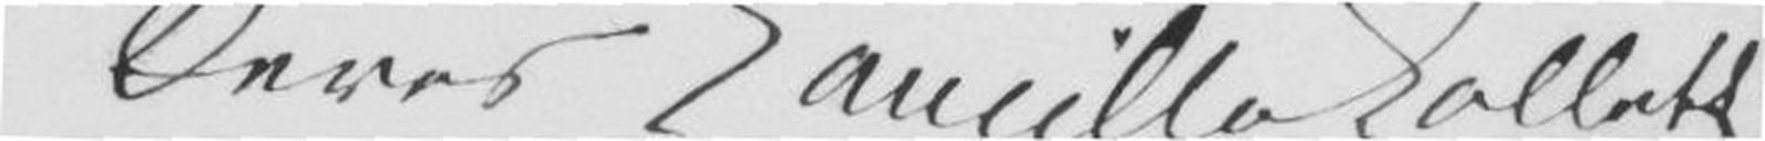Deres Camilla Collett
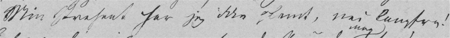Min Present har jeg ikke glemt, nei langtfra!
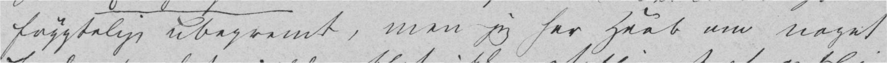frygteligt ubeqvemt, men jeg har Haab om noget
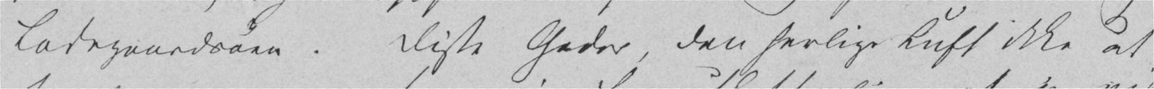Ladegaardsøen. Disse Goder, den herlige Luft ikke at
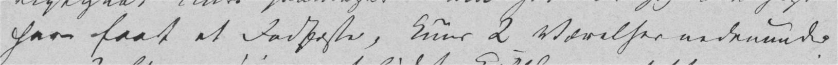have faat et Fodfæste, kuns 2 Værelser nedenunder
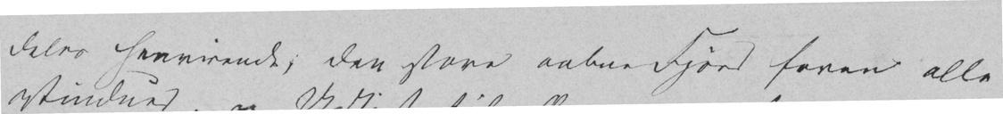henrivende, den store aabne Fjord foran alle
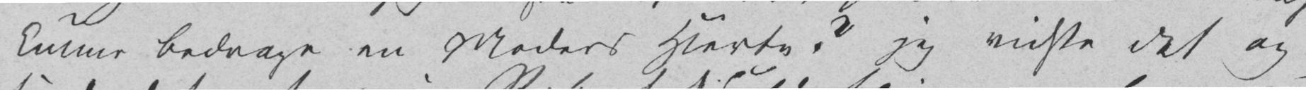kunne bedrage en Moders Hjerte? jeg vidste det og
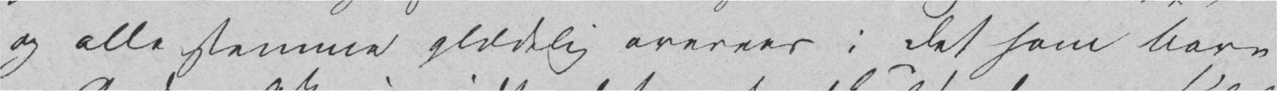og alle stemme glædelig overens i det som bare
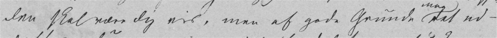Den skal være Dig vis, men af gode Grunde det ud-
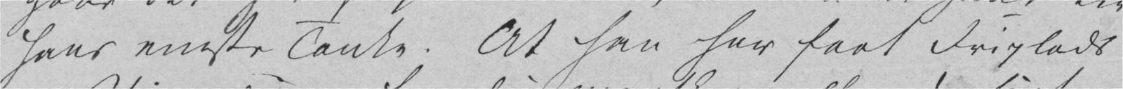hans eneste Tanke. At han har faat Friplads
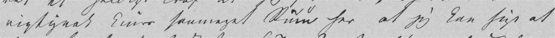rigtignok kuns saameget Rum her at jeg kan sige at
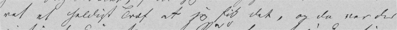ret et heldigt Træf at jeg fik det, og da var der
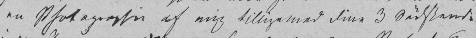en Photographie af mig tillige med Dine 3 Søskende
